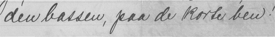den bassen, paa de korte ben!
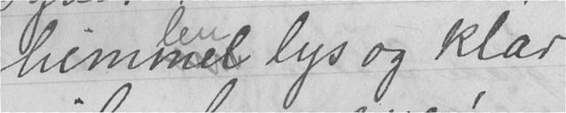himlen lys og klar
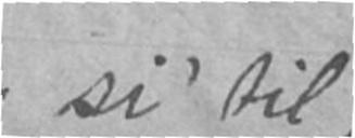si' til
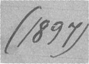(1897)
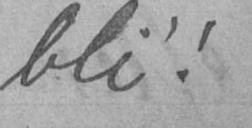bli'!
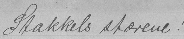Stakkels stærene!
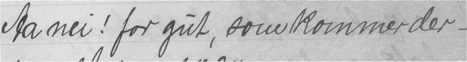Aa nei! for gut, som kommer der -
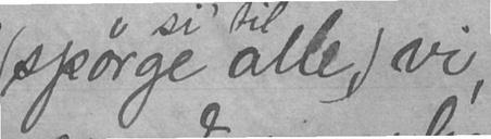(spørge alle,) vi,
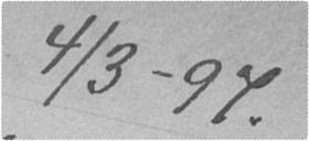4/3-97.
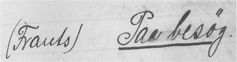(Frants) Paa besøg.
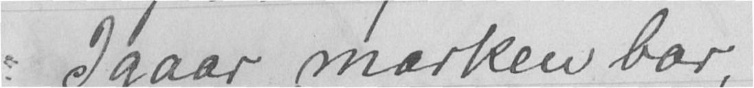I gaar marken bar,
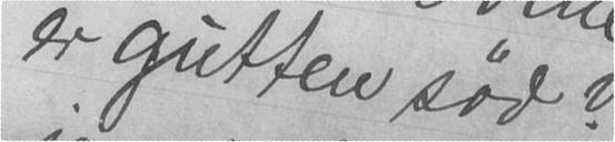er gutten sød?
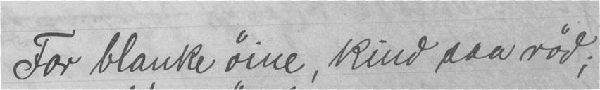For blanke øine, kind saa rød;
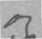og
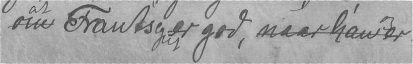om Frantsegut er god, naar han er
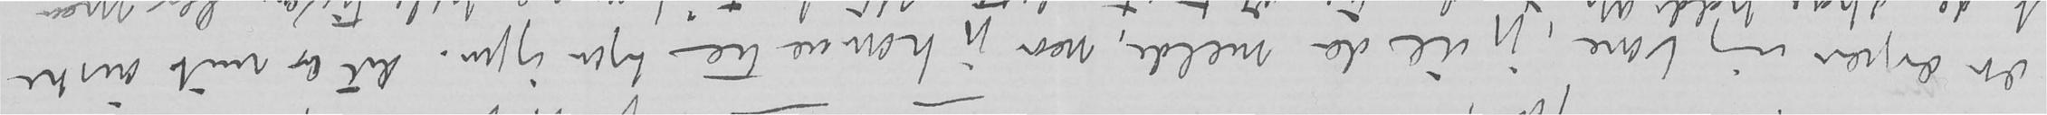det ærgrer mig bare, jeg vil da melde, naar jeg komme til byen igjen. Det er mit ønske
